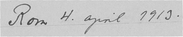Rom 4. april 1913.
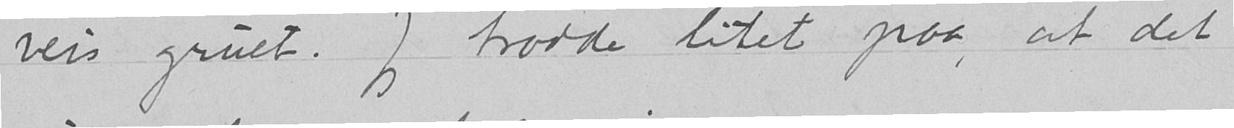veis gruet. Jeg trodde litet paa, at det
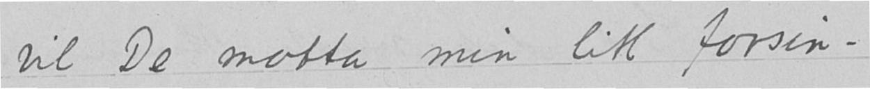vil De motta min litt forsin-
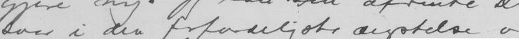svar i den forfærdeligste ængstelse og
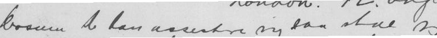Dersom De kan Assistere mig saa skal jeg
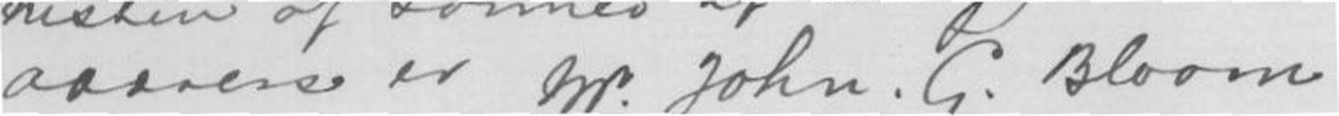addresse er Mr. John. G. Blomm
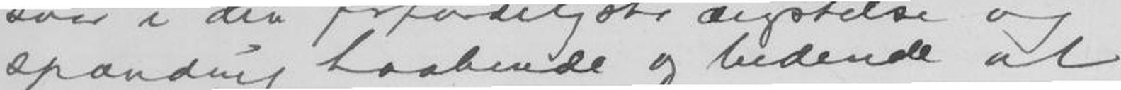spændning haabende og bedende at
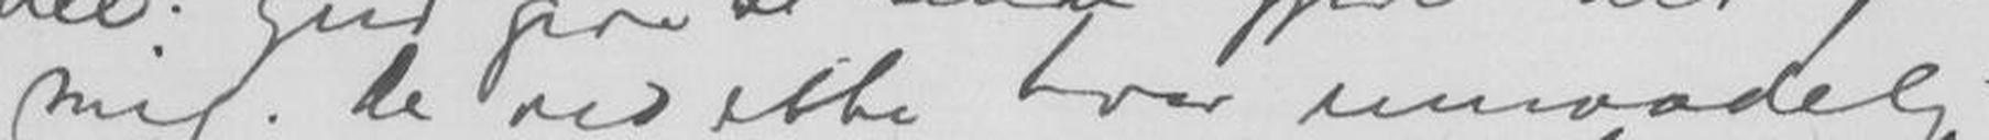for mig. De ved ikke hvor umaadelig
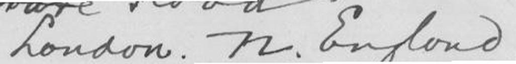London N. England.
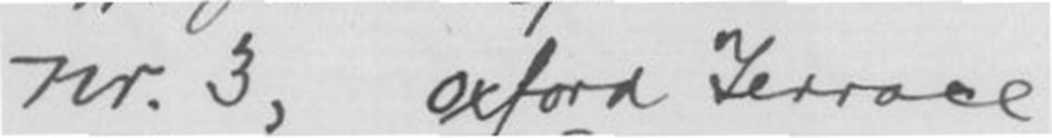Nr. 3 Oxford Terrace
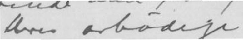Deres ærbødige
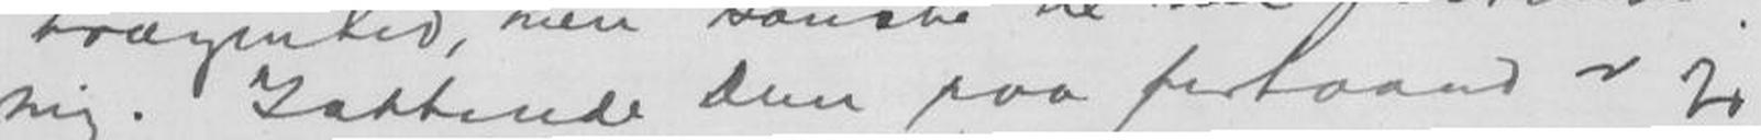mig. Takkende Dem paa forhaand er jeg
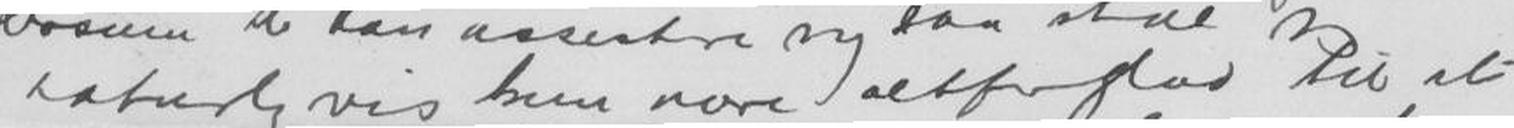naturligvis kun være altforglad til at
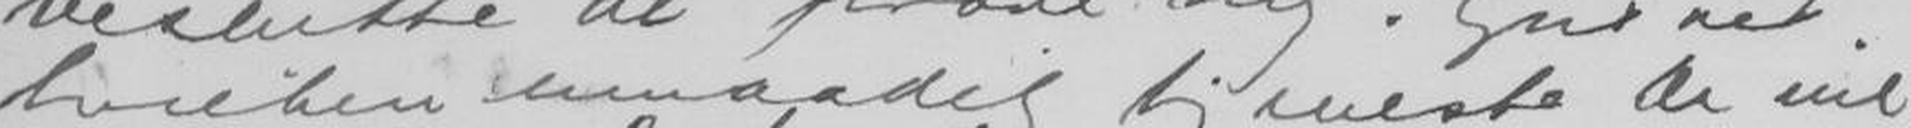hvilken umaadelig tjeneste De vil
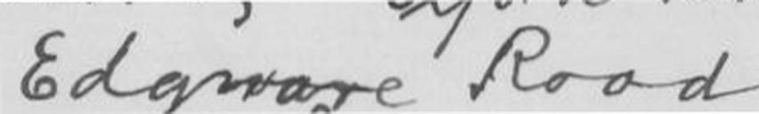Edgware Rood
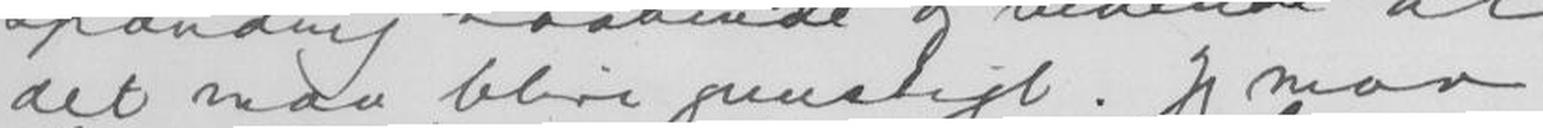det man bliv gunstigt. Jeg maa
